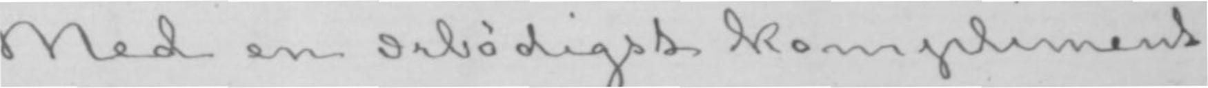Med en ærbødigst kompliment
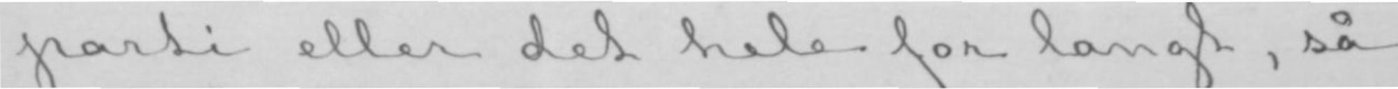parti eller det hele for langt, så
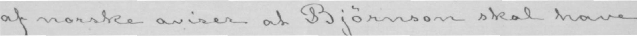af norske aviser at Bjørnson skal have
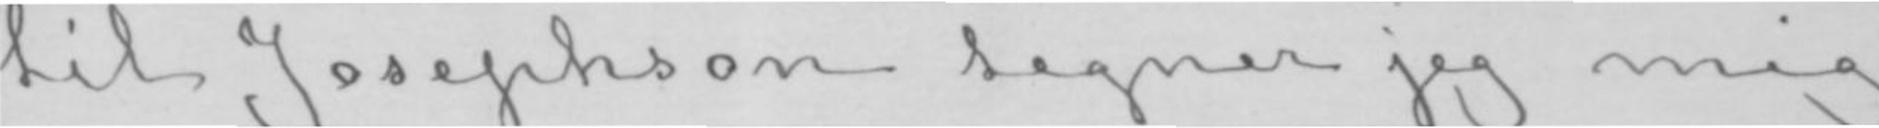til Josephson tegner jeg mig
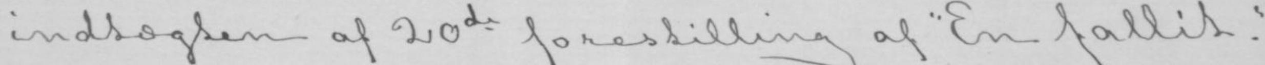indtægten af 20de forestilling af «En fallit.»
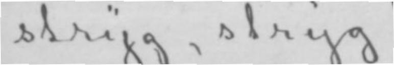stryg, stryg!
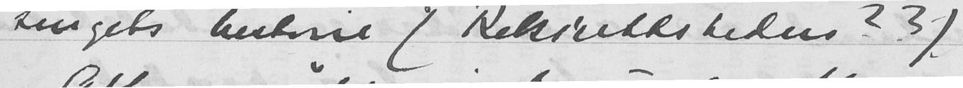tingets historie (Riksrettstidende 23)
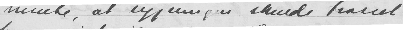mente, at regjeringen skulde trasset
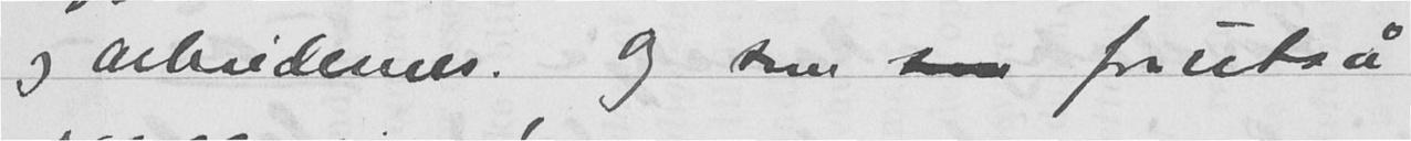og arbeidernes. Og som  forutså
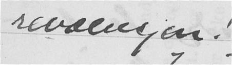revolusjon!
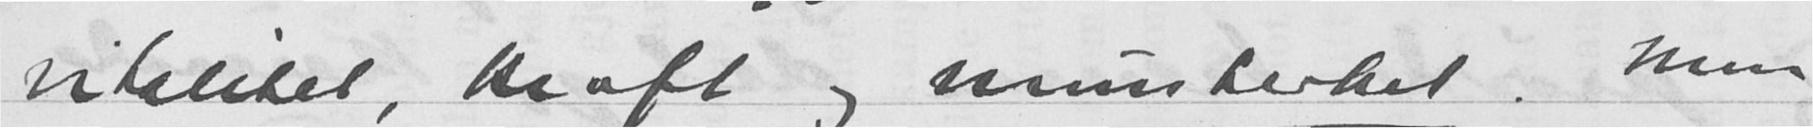vitalitet, kraft og munterhet. Men
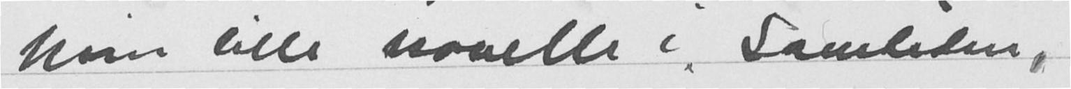Min lille novelle i Samtiden,
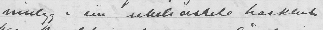innlegg i sin ubeherskete kaskleit
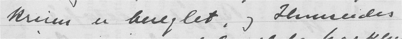krisen er beseglet, og Hunseides
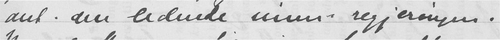ant. den ledende umin i regjeringen.
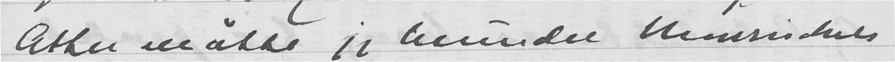Atter måtte jeg beundre ministerens
